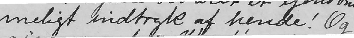meligt indtryk af hende! Og
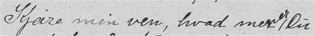Kjære min ven, hvad mener Du
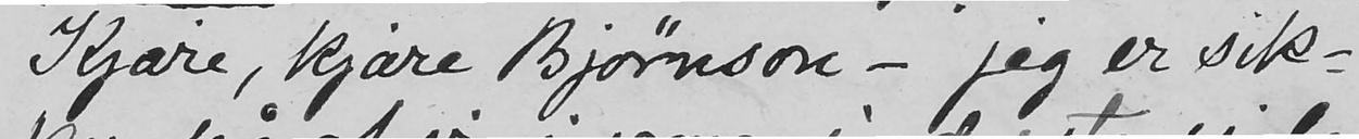Kjære, Kjære Bjørnson - Jeg er sik-
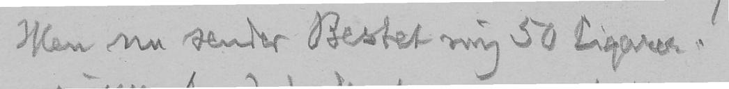Men nu sender Bestet mig 50 Cigarer!
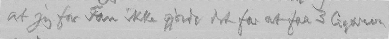at jeg for Fan ikke gjorde det for at faa 3 Cigarer
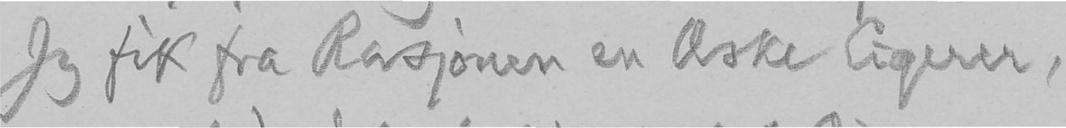Jeg fik fra Rasjonen en Æske Cigerer,
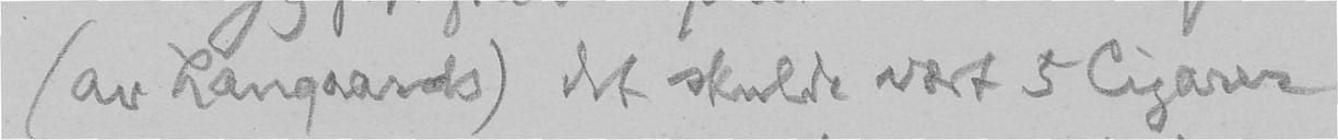(av Langaards) det skulde vært 5 Cigarer
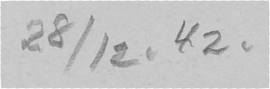28/12. 42.
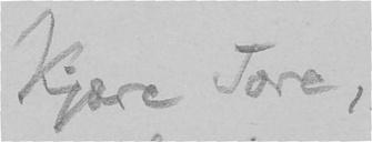Kjære Tore,
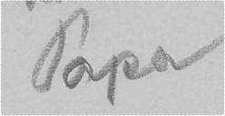Papa
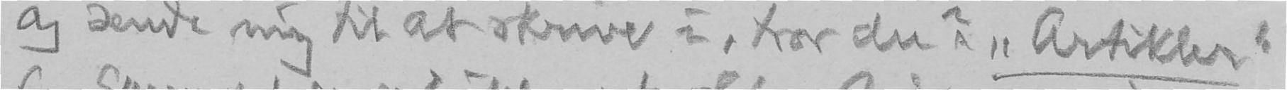og sende mig til at skrive i, tror du? "Artikler"
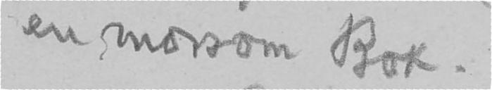en morsom Bok.
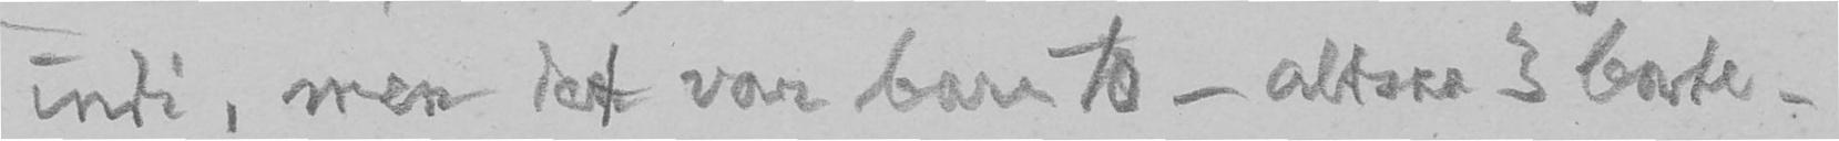indi, men det var bare to - altsaa 3 borte.
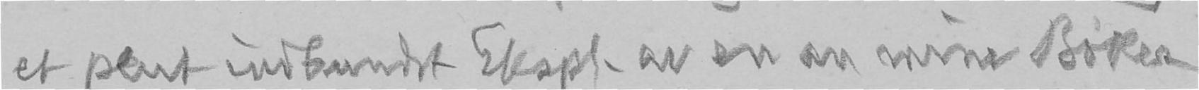et pent indbundet Ekspl. av en av mine Bøker
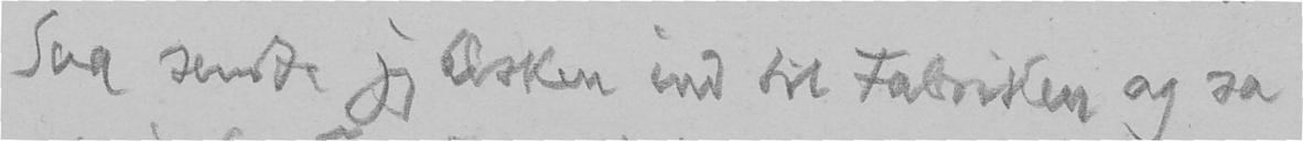Saa sendte jeg Æsken ind til Fabriken og sa
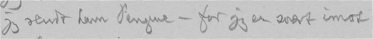jeg sendt dem Pengene - for jeg er svært imot
